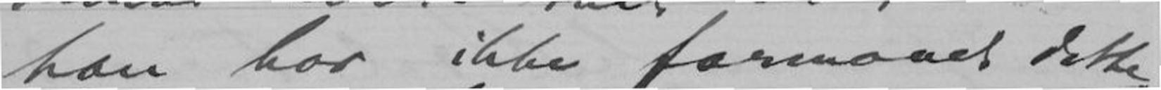han har ikke formaaet dette
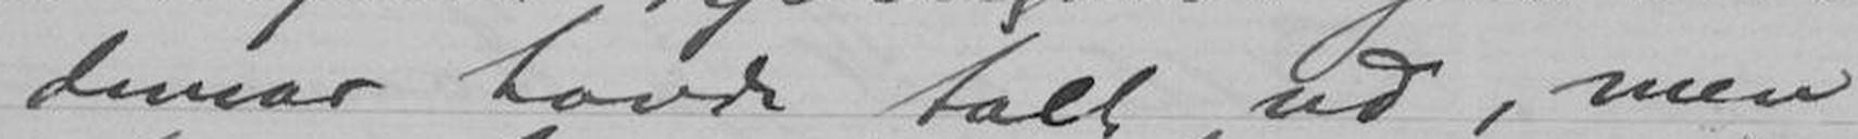demar havde talt ud, men
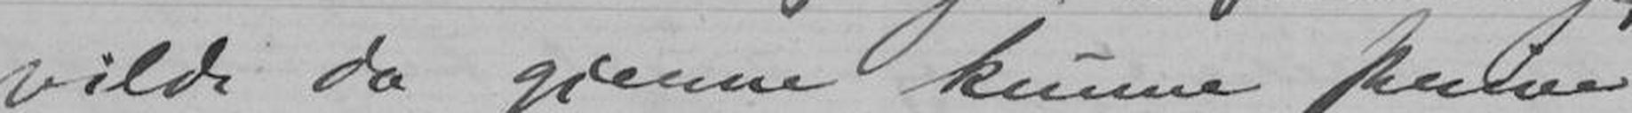vilde da gjerne kunne skrive
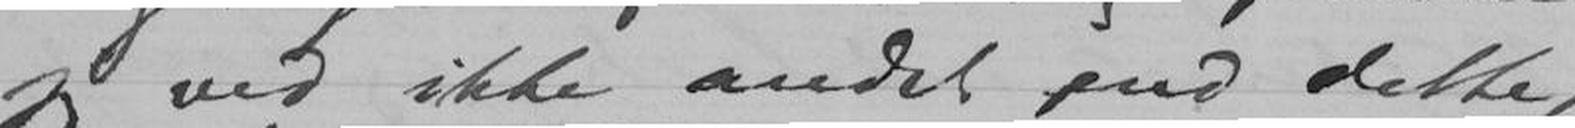jeg ved ikke andet end dette,
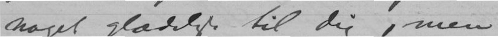noget glædeligt til Dig, men
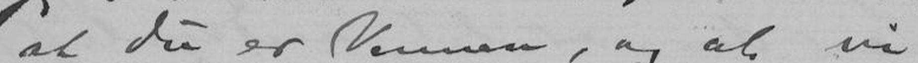at Du er Vennen, og at vi
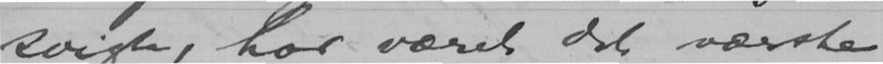svigte, har været det værste
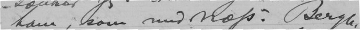ham, som med Næss. Bergh,
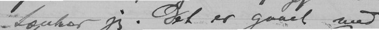tænker jeg. Det er gaaet med
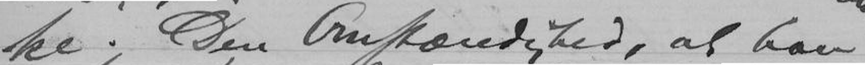ke. Den Omstændighed, at han
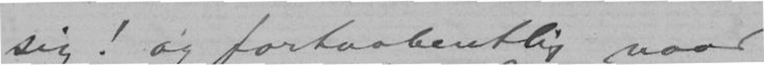sig! og forhaabentlig naar
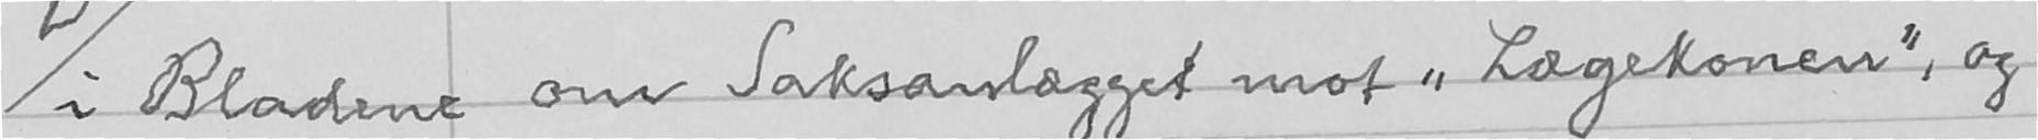i Bladene om Saksanlægget mot "Lægekonen", og
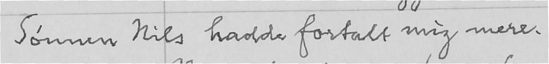Sønnen Nils hadde fortalt mig mere.
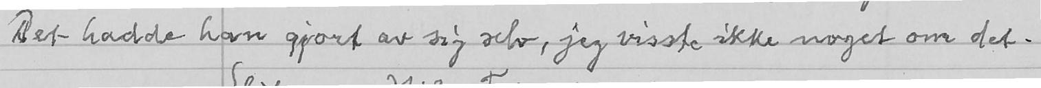Det hadde han gjort av sig selv, jeg visste ikke noget om det.
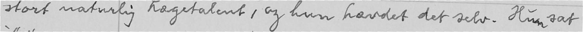stort naturlig Lægetalent, og hun hævdet det selv. Hun sat
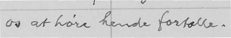os at høre hende fortælle.
